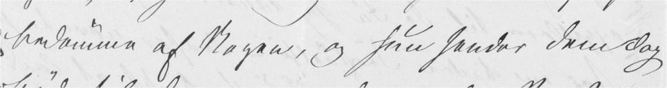bedømme af Nogen, og hun sender dem dog
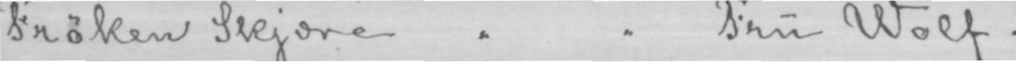Frøken Skjære " " Fru Wolf.
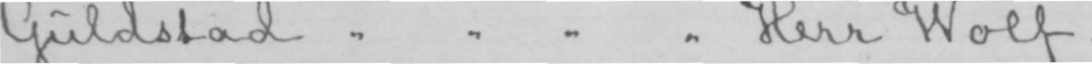Guldstad " " "  " Herr Wolf.
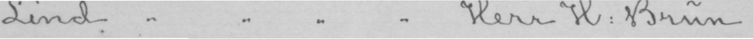Lind " " "  " Herr H: Brun.
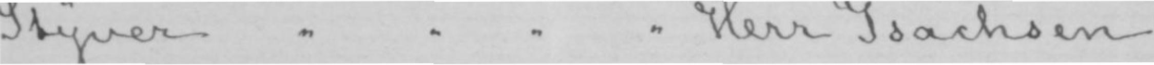Styver " " "  " Herr Isachsen.
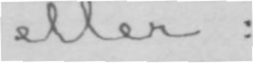eller:
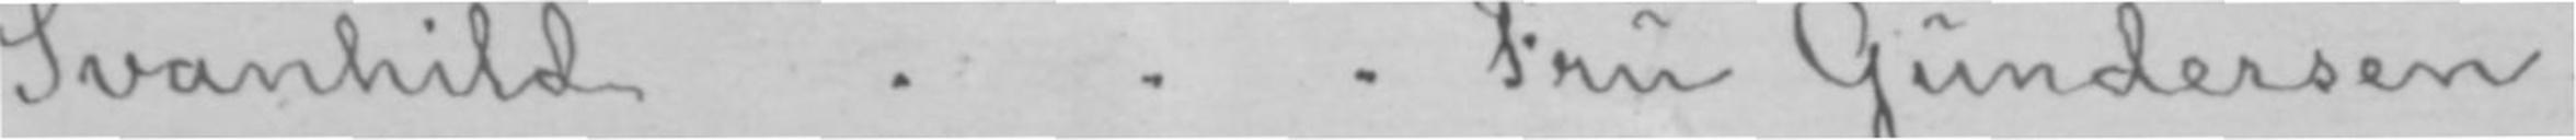Svanhild " " " Fru Gundersen.
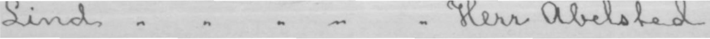Lind  " " " " " Herr Abelsted.
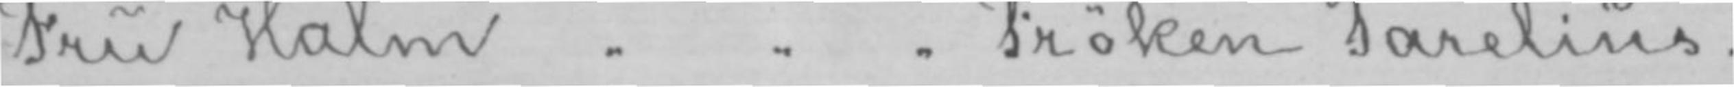Fru Halm " " " Frøken Parelius.
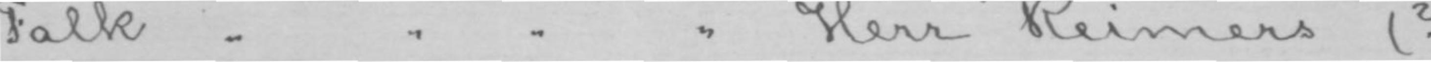Falk " " "  " Herr Reimers (?)
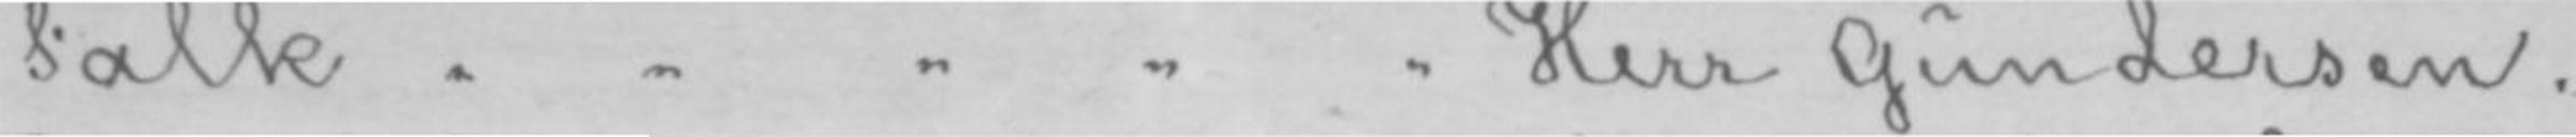Falk  " " " " " Herr Gundersen.
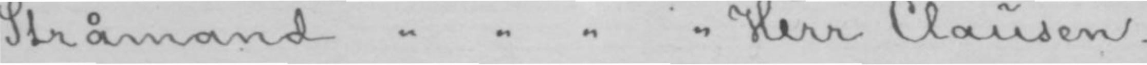Stråmand " " "  " Herr Clausen.
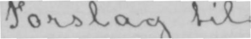Forslag til
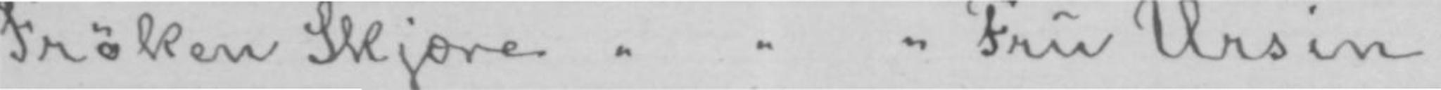Frøken Skjære " " " Fru Ursin.
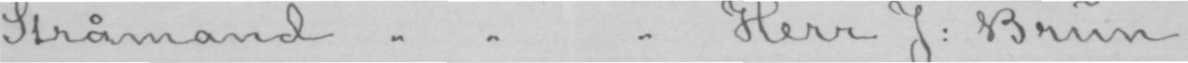Stråmand " " " Herr J: Brun.
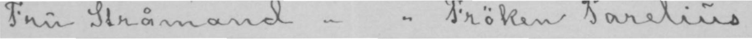Fru Stråmand " " Frøken Parelius.
